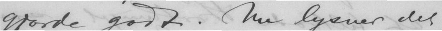gjorde godt. Nu lysner det,
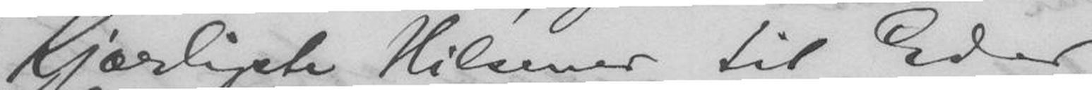Kjærligste Hilsener til Eder
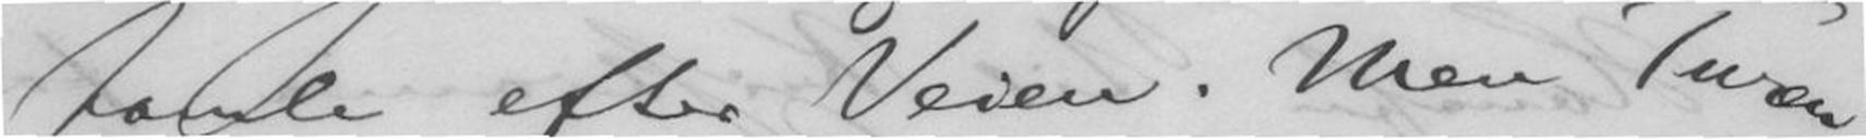famle efter Veien. Men Turen
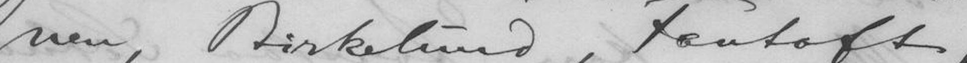nen. Birkelund, Fantoft,
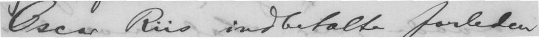Oscar Riis indbetalte forleden
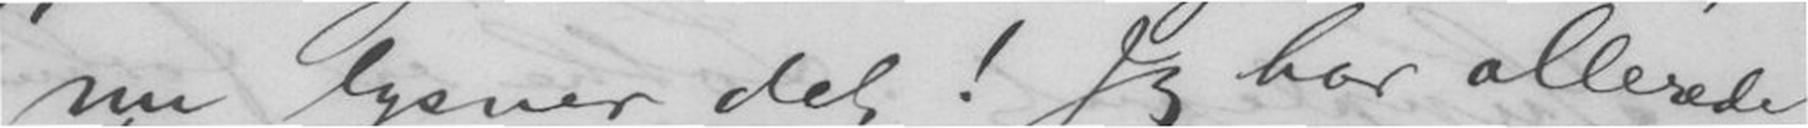nu lysner det! Jeg har allerede
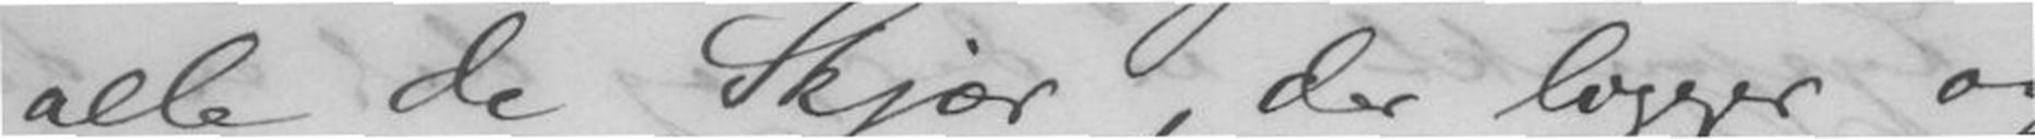alle de Skjær, der ligger og
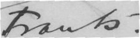Frants.
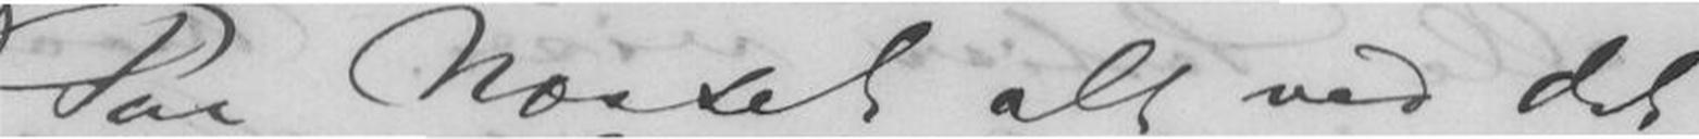Paa Næsset alt ved det
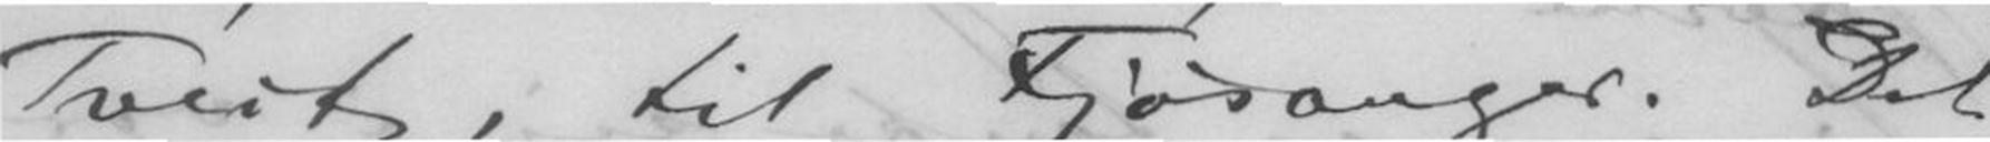Tveit, til Fjøsanger. Det
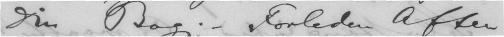Din Bog. - Forleden Aften
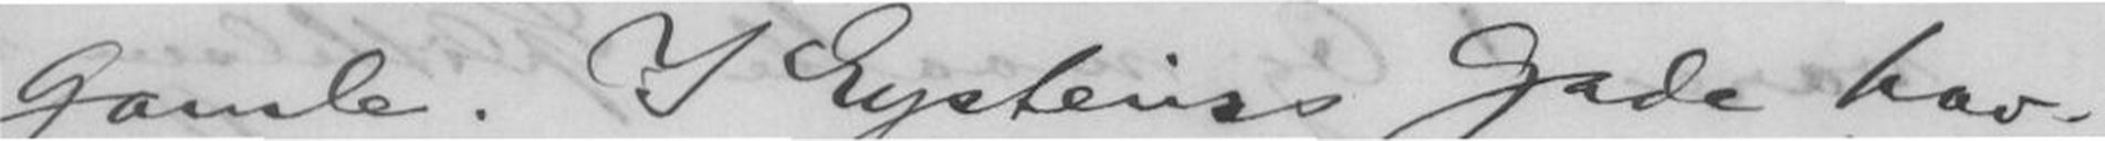gamle. I Eysteins Gade hav-
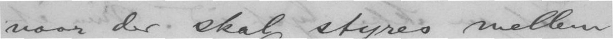naar der skal styres mellem
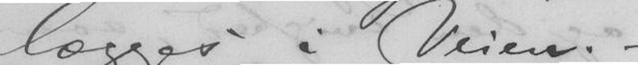lægges i Veien. -
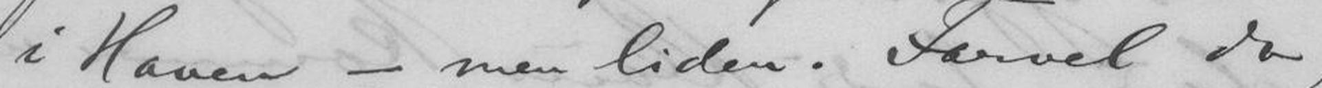i Haven - men liden. Farvel da,
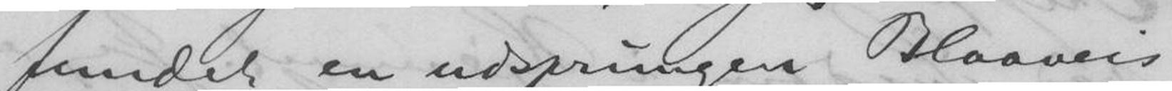fundet en udsprunget Blaavei
